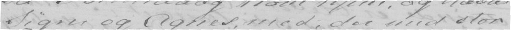Signe og Agnes med, der med stor
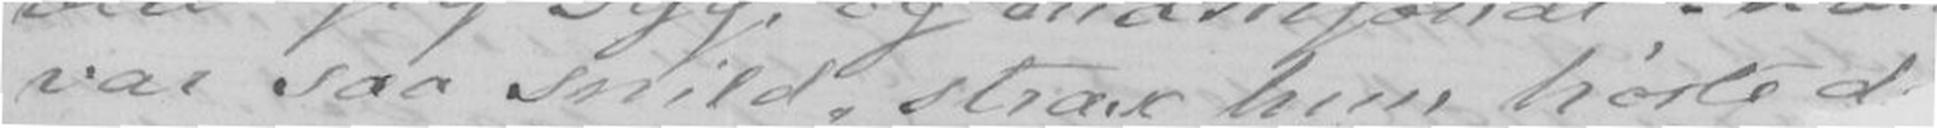var saa snild strax hun hørte d
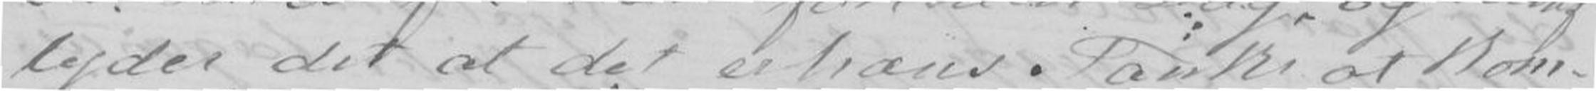lyder det at det er hans Tanke at kom-
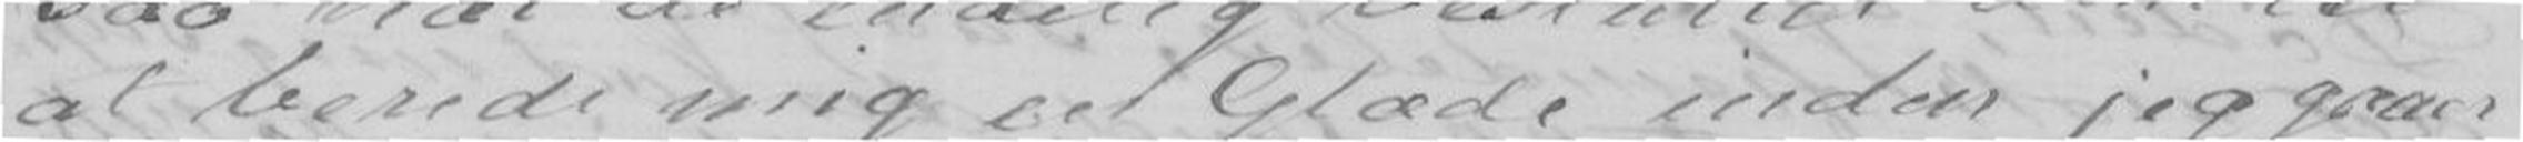at berede mig en Glæde inden jeg gaaer
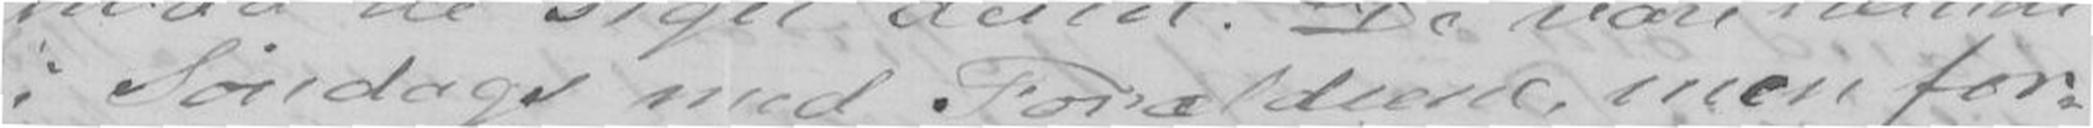i Søndags med Forældrene, men for-
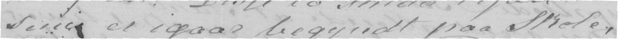sene er igaar begyndt paa Skole,
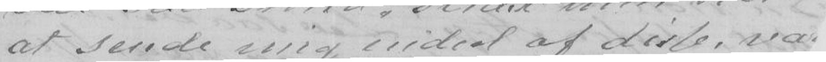at sende mig endeel af disse va
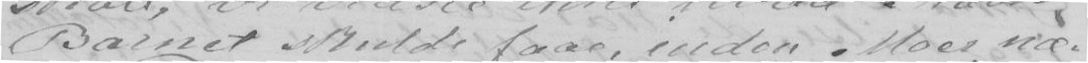Barnet skulde faae, inden Moer næ-
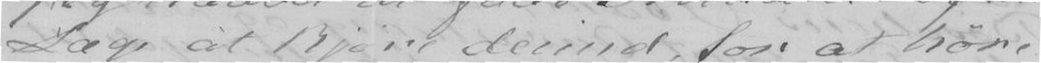Læge at kjøre derind, for at høre
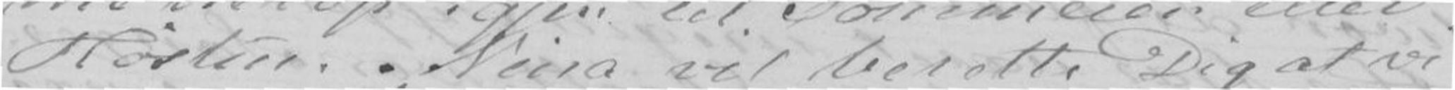Høsten. Nina vil berette Dig at vi
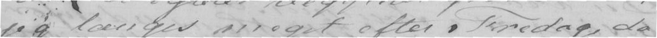jeg længes meget efter Fredag da
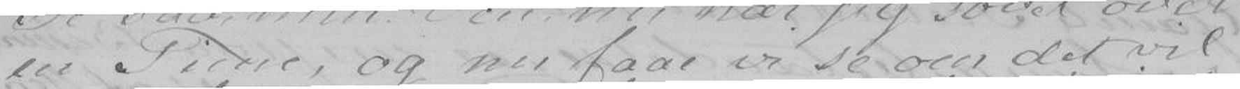en Time, og nu faar vi se om det vil
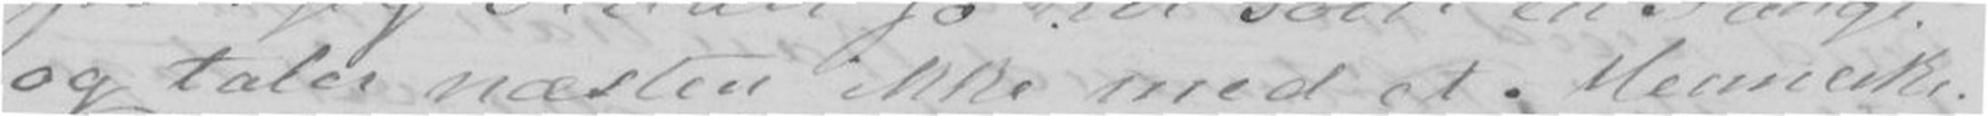og taler næsten ikke med et Menneske.
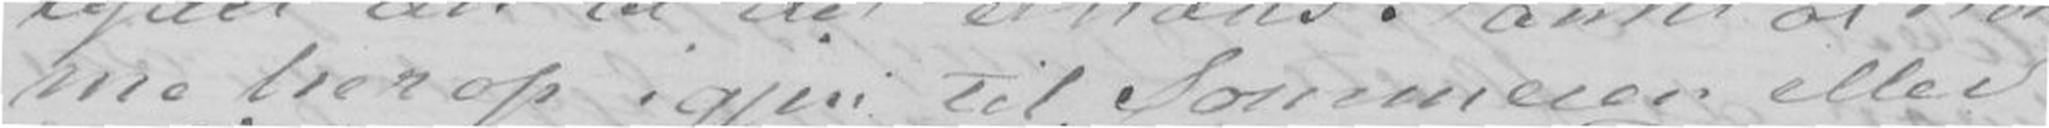me herop igjen til Sommeren eller
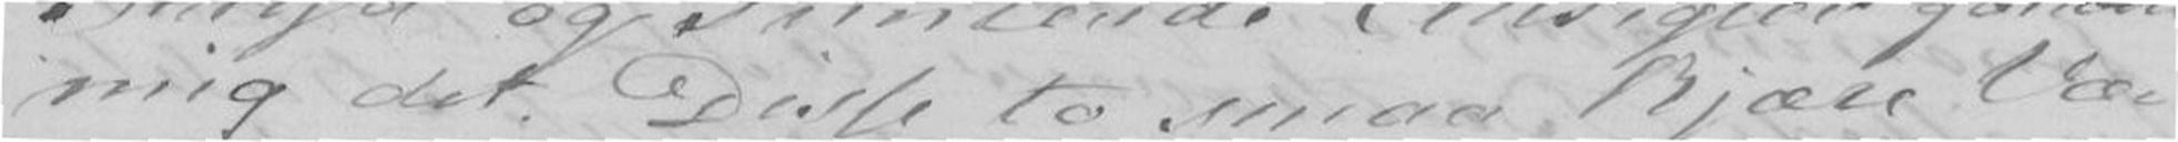mig det Disse to smaa kjære Væ-
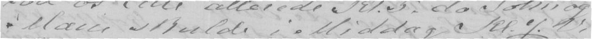Marie Marie skulde i Middag Kl.7. Vi
